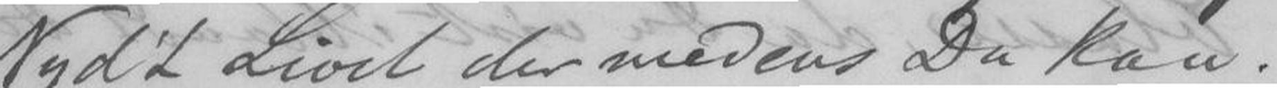Nyd't Livet der medens Du kan.
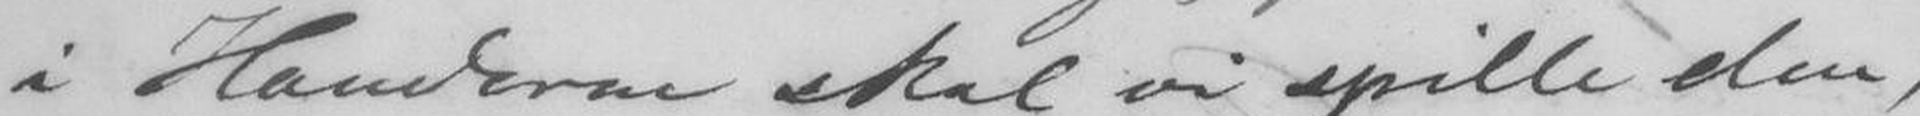i Hænderne skal vi spille den
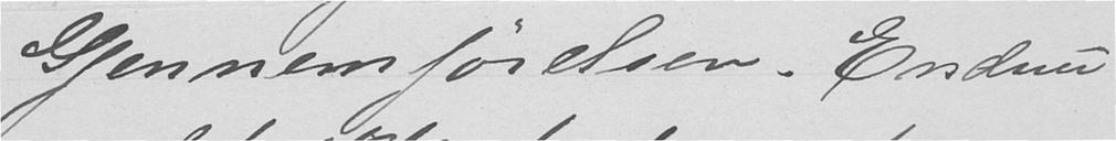Gjennemførelsen. Endnu
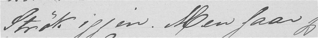Strøk igjen. Men faar jeg
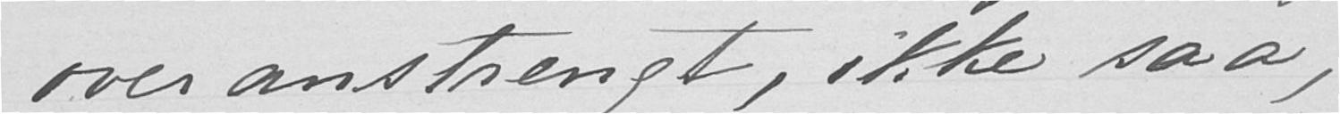overanstrengt, ikke saa,
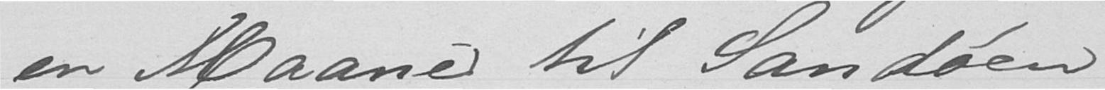en Maaned til Sandøen
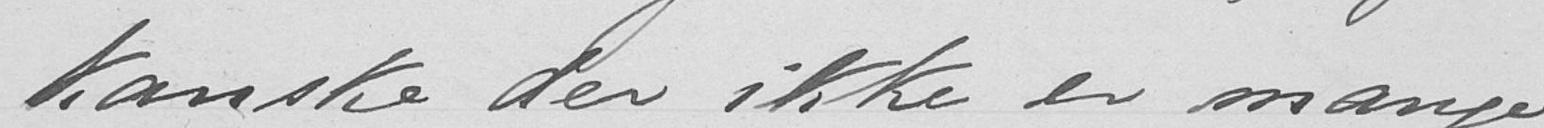kanske der ikke er mange
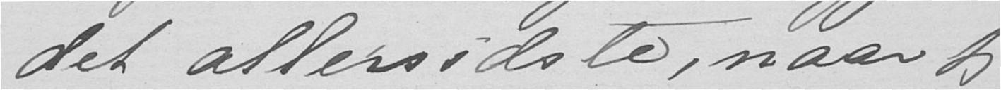det allersidste, naar jeg
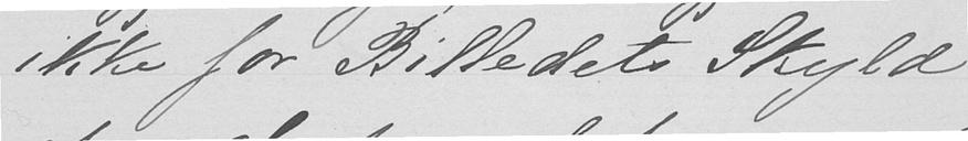ikke for Billedets Skyld
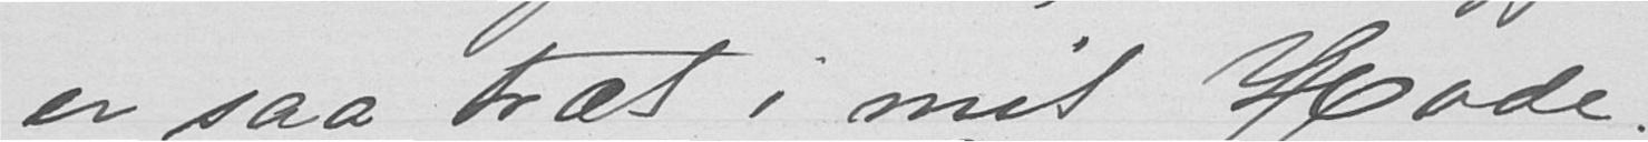er saa træt i mit Hode.
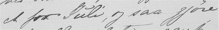et for Juli, og saa gjøre
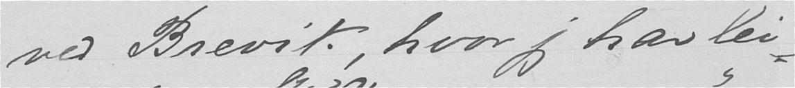ved Brevik, hvor jeg har lei-
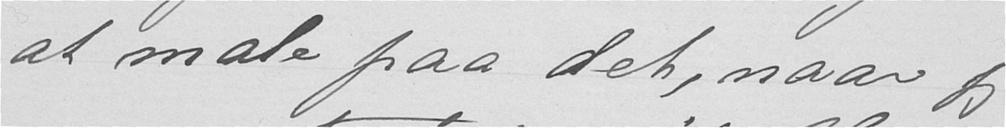at male paa det, naar jeg
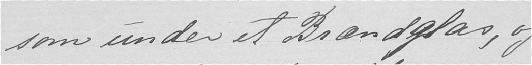som under et Brændglas, og
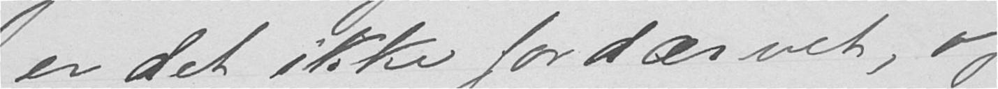er det ikke fordærvet, og
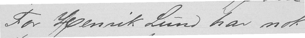For Henrik Lund har nok
